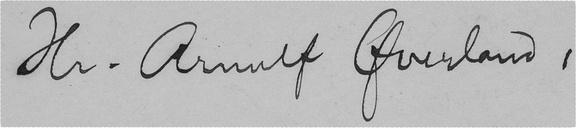Hr. Arnulf Øverland,
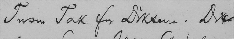Tusen Tak for Diktene. Det
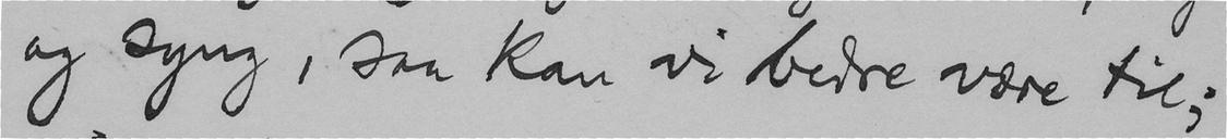og syng, saa kan vi bedre være til;
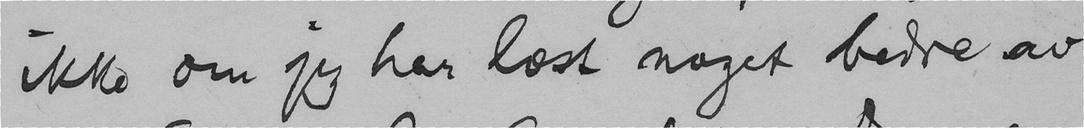ikke om jeg her læst noget bedre av
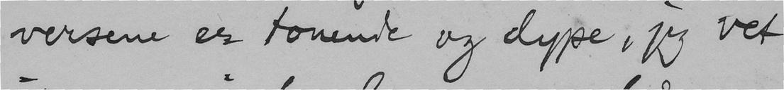versene er tonende og dype, jeg vet
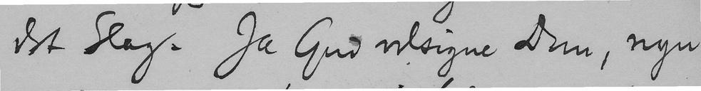det Slag. Ja Gud velsigne Dem, nyn
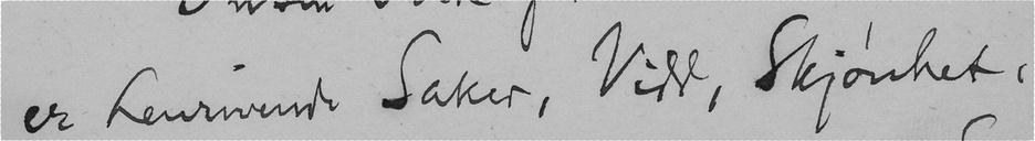er henrivende Saker, Vidd, Skjønhet,
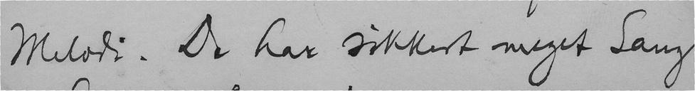Melodi. De har sikkert meget Sang
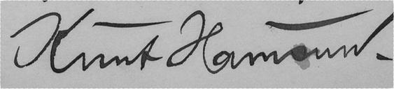Knut Hamsun.
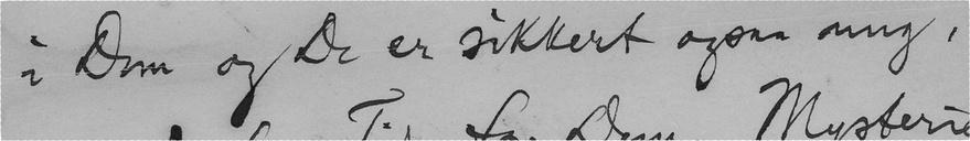i Dem og De er sikkert ogsaa ung,
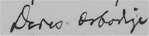Deres ærbødige
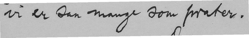vi er saa mange som prater.
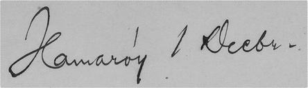Hamarøy 1 Decbr.
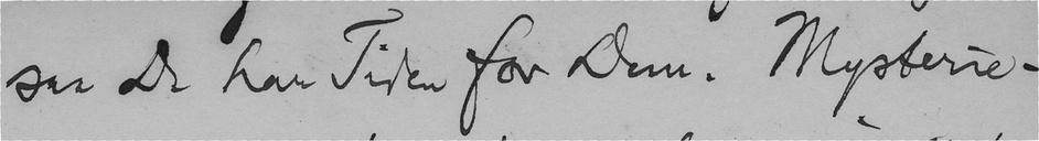saa De har Tiden for Dem. Mysterie-
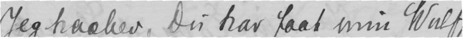Jeg haaber Du har faat min Wulffie
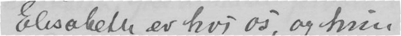Elisabeth er hos os, og hun
